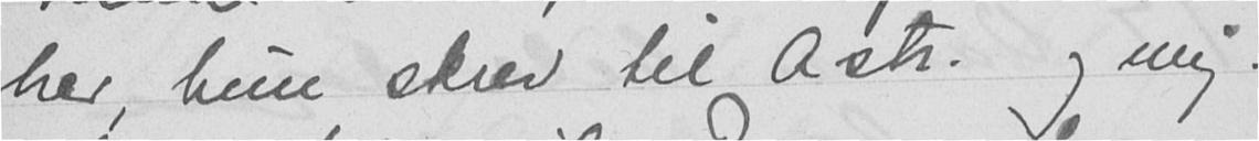brev, hun skrev til Astr. og mig.
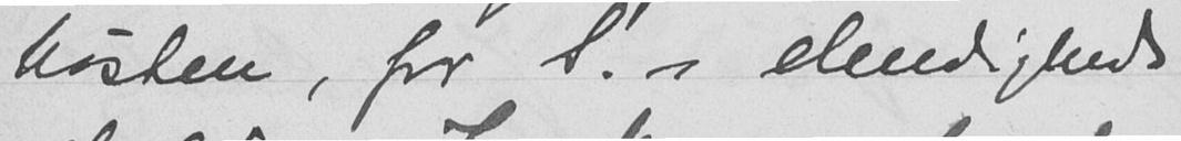høsten, for S.s elendigheds
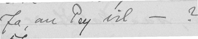Ja, om Pey vil - ?
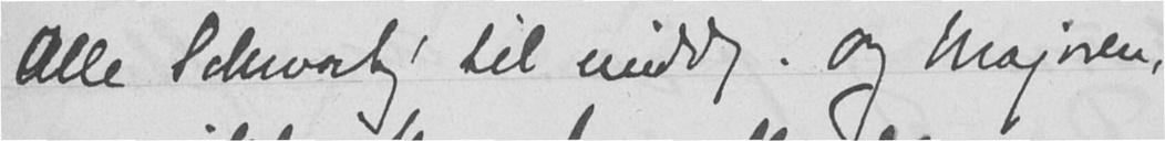Alle Schwartz' til middag. Og Majoren,
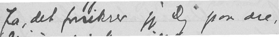Ja, det forsikrer jeg Dig paa ære,
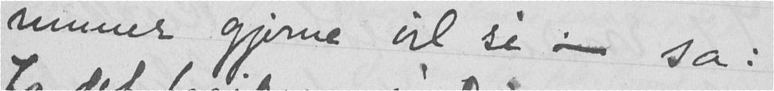immer gjerne vil si - sa:
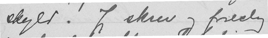skyld. Jeg skrev og foreslog
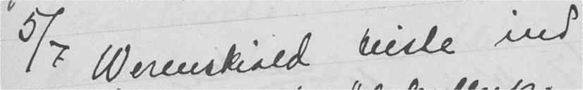5/7 Werenskold reiste ind
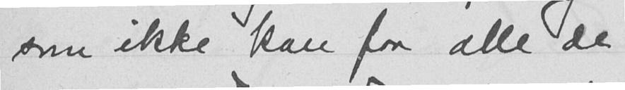som ikke kan faa alle de
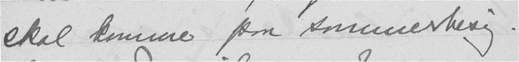skal komme paa sommerbesøg.
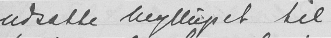udsætte bryllupet til
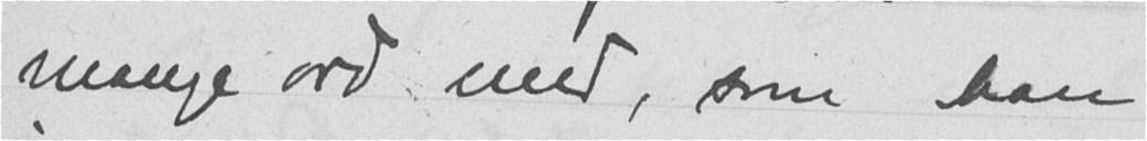mange ord med, som han
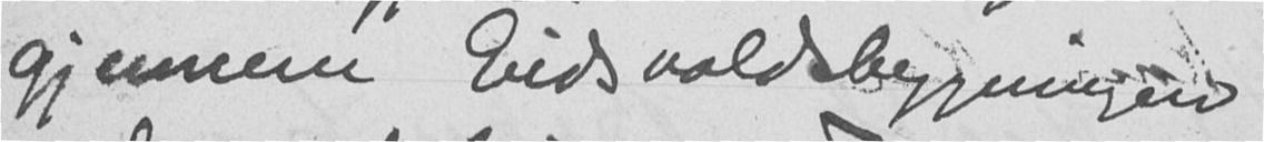gjennem Eidsvoldsbygningen
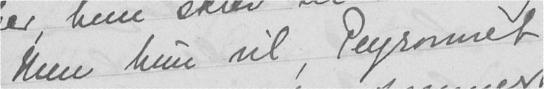Men hun vil, Peyronnet
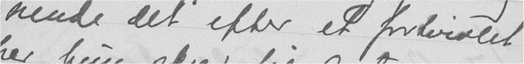hende det efter et fortvivlet
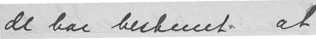de har bestemt at
